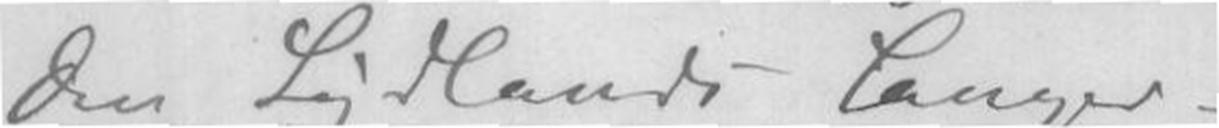den Sydlands Sanger -
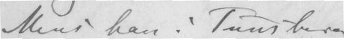Mens han i Tunsberg
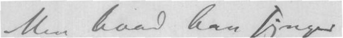Men hvad han sjunger,
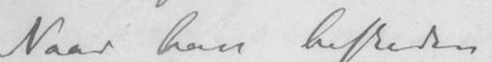Naar han beskeden
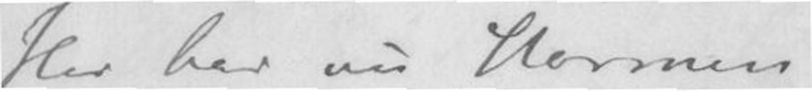Her har nu Stormen
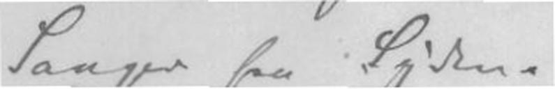Sanger fra Syden.
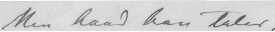Men hvad han taler,
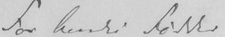for hendes fødder
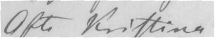Ofte Kristina
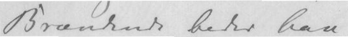Brændende bedre han -
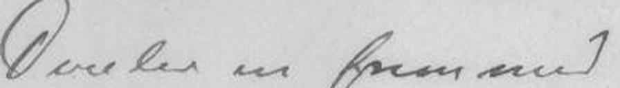Dveler en fremmed
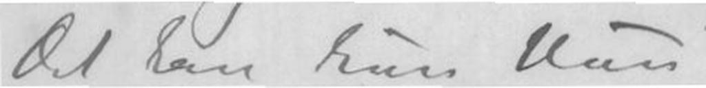Det kan kun Hun
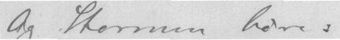Og Stormen høre.
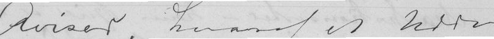Aviser, hvoraf et Uddrag
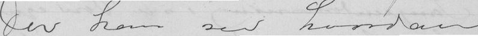du kan see hvordan
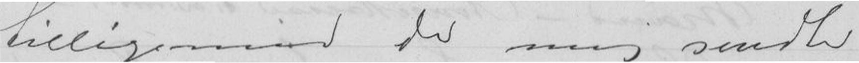tilligemed de mig sendte
